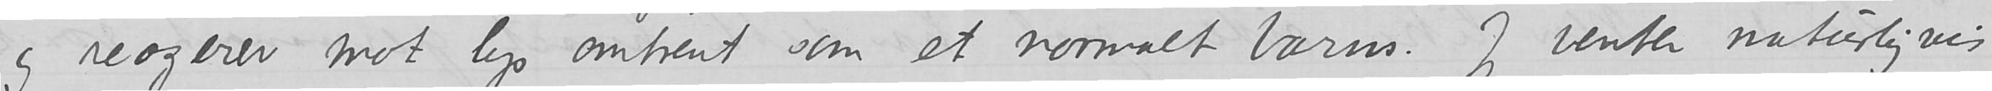og reagerer mot lys omtrent som et normalt barns. Jeg venter naturligvis
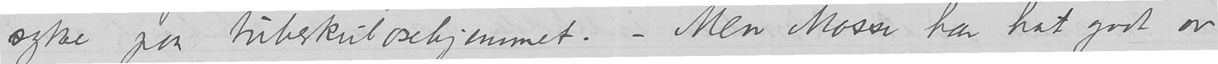syke paa tuberkulosehjemmet. – Men Mosse har hat godt av
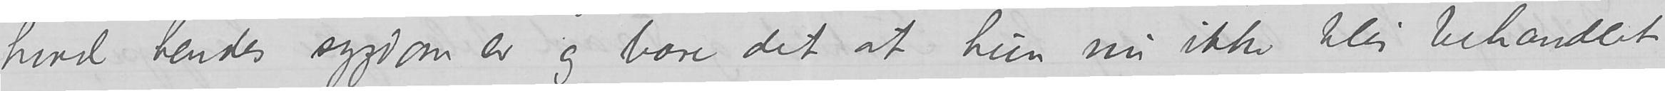hvad hendes sygdom er og bare det at hun nu ikke blir behandlet
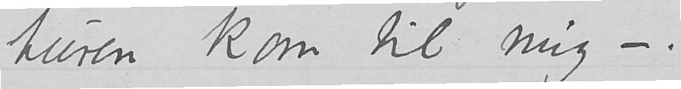turen kom til mig -.
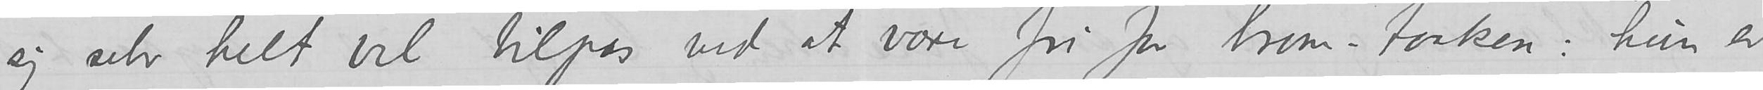sig selv helt vel tilpas ved at være fri for brom-taaken hun er
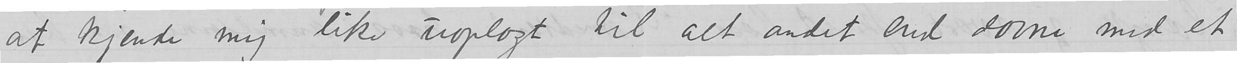at kjende mig like uoplagt til alt andet end dovne med et
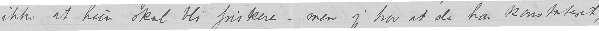ikke at hun skal bli friskere – men jeg tror at de har konstateret,
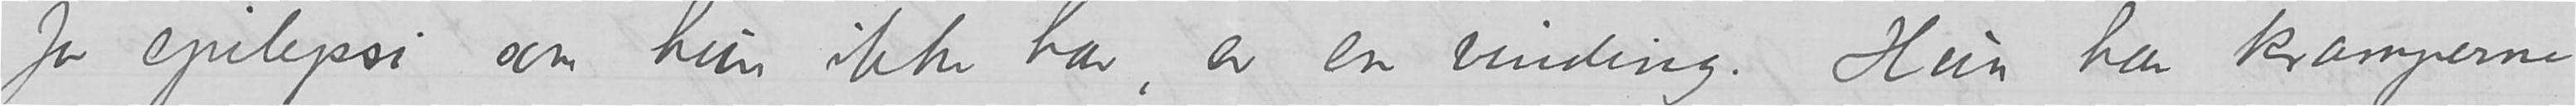for epilepsi som hun ikke har, er en vinding. Hun har kramperne
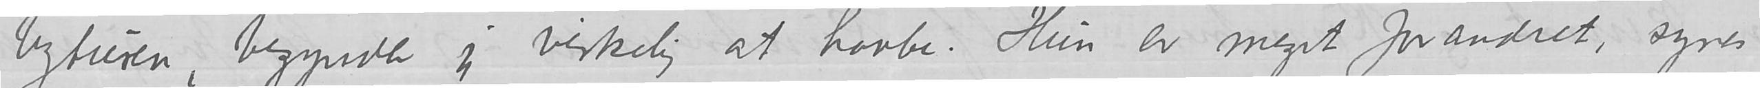byturen, begyndte jeg virkelig at haabe. Hun er meget forandret, synes
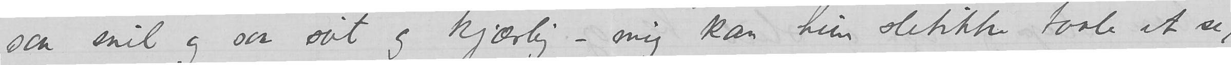saa snil og saa søt og kjærlig – mig kan hun sletikke taale at se,
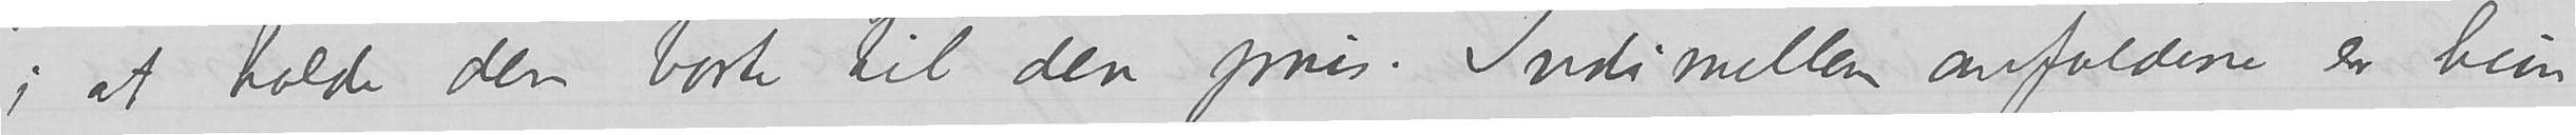i at holde dem borte til den pris. Indimellem anfaldene er hun
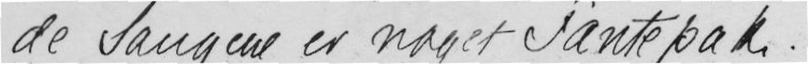de Sangene er noget Fantepak.
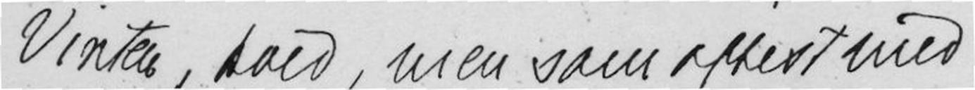Vinter, old, men som oftest med
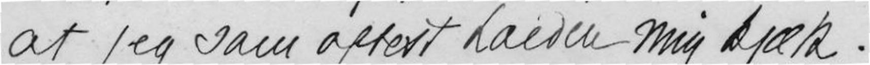at jeg som oftest holder mig kjæk.
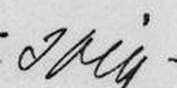svig-
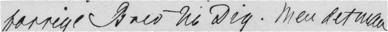forrige Brev til Dig. Men det maa
